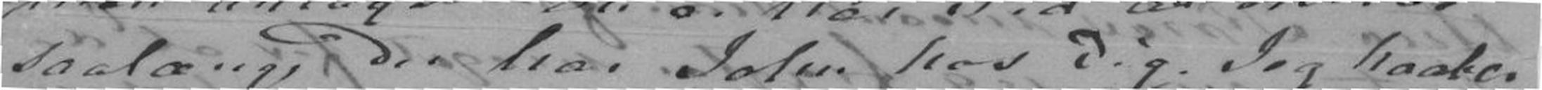saalenge Du har John hos Dig. Jeg haaber
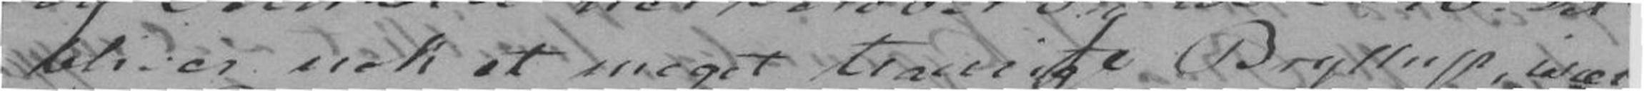bliver nok et meget traurigt Bryllup, især
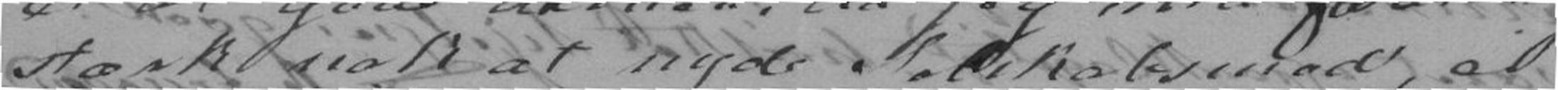stærk nok at nyde Selskabsmad, ei
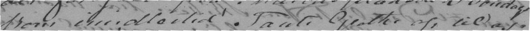kom imidlertid Tante Grethe op til os
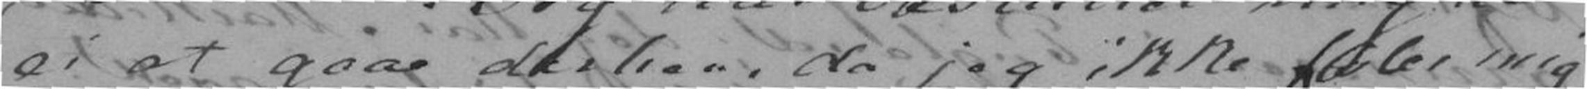ei at gaae derhen, da jeg ikke føler mig
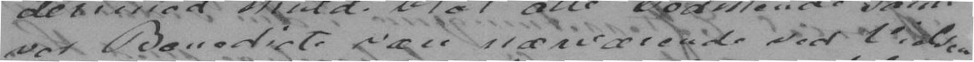vor Benedicte være nærværende ved Vielsen
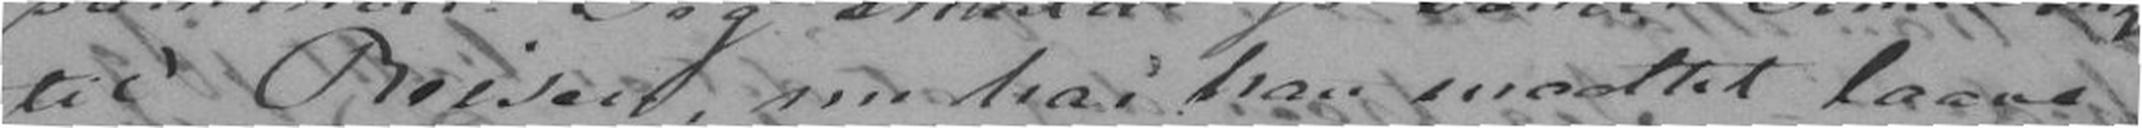til Reisen, men har han maattet laane
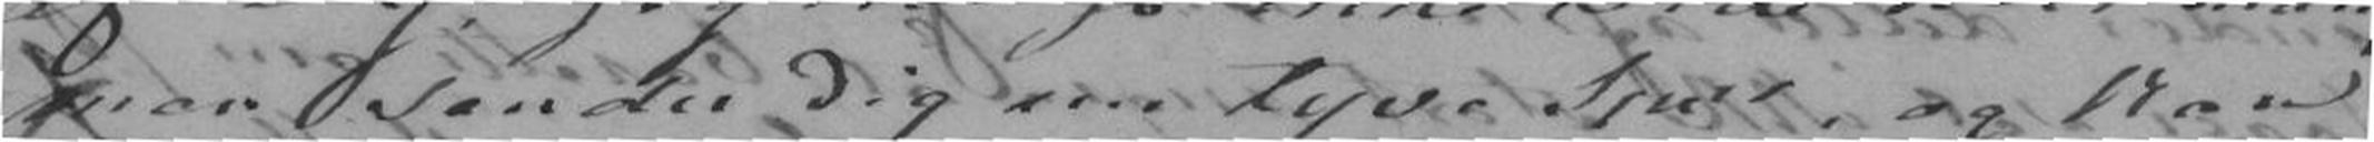men sender Dig nu tyve Spd., og kan
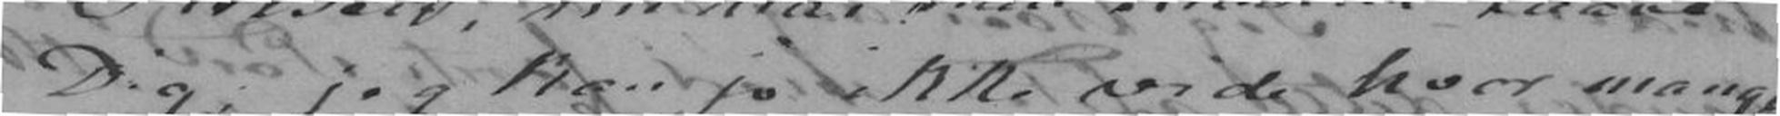af Dig, jeg kan jo ikke vide hvor mange,
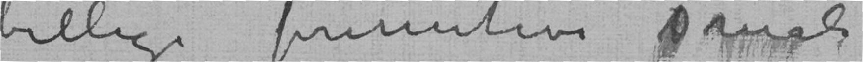billige primitive smale
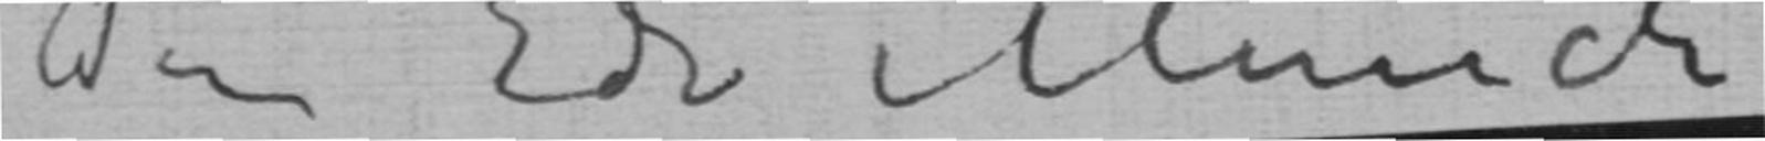Din Edv Munch
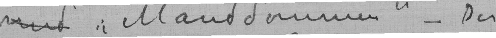med «Manddommen» – Der
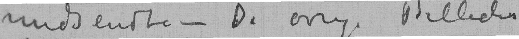medsendte – De øvrige Billleder
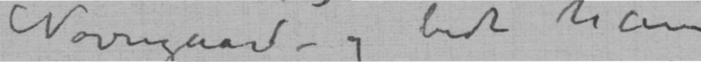Nørregaard – og bedt ham
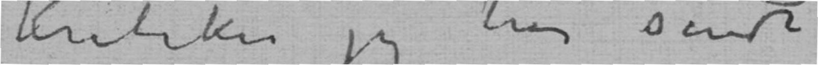Kritiker jeg har sendt
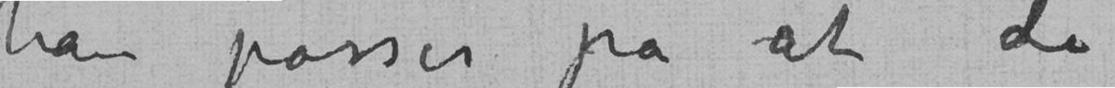han passer på at de
